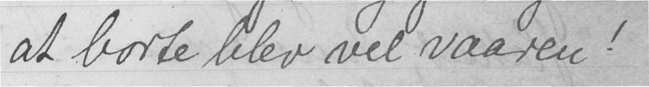at borte blev vel vaaren!
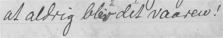at aldrig blir det vaaren!
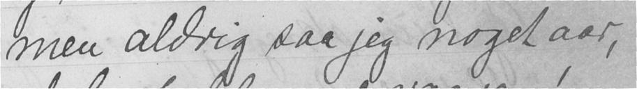men aldrig saa jeg noget aar,
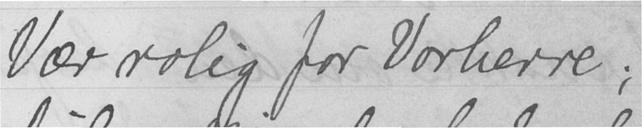Vær rolig for Vorherre;
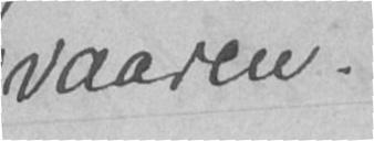vaaren.
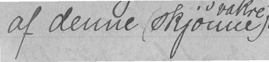af denne (skjønne)
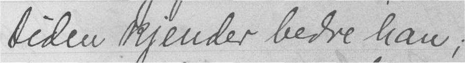tiden kjender bedre han;
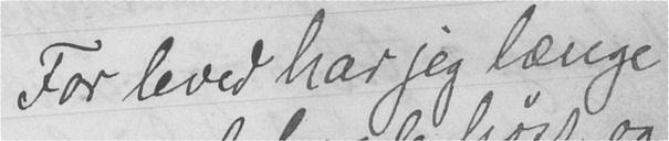For leved har jeg længe
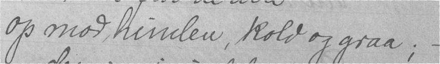op mod himlen, kold og graa; -
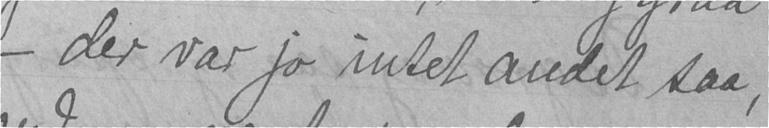- der var jo intet andet saa,
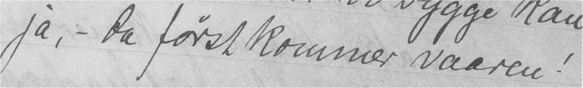ja, - da først kommer vaaren!
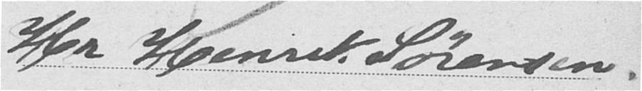Hr Henrik Sørensen.
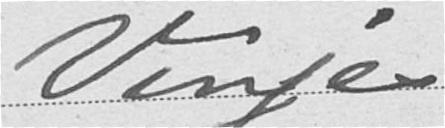Vinje
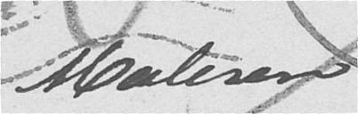Maleren
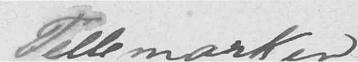Tellemarken
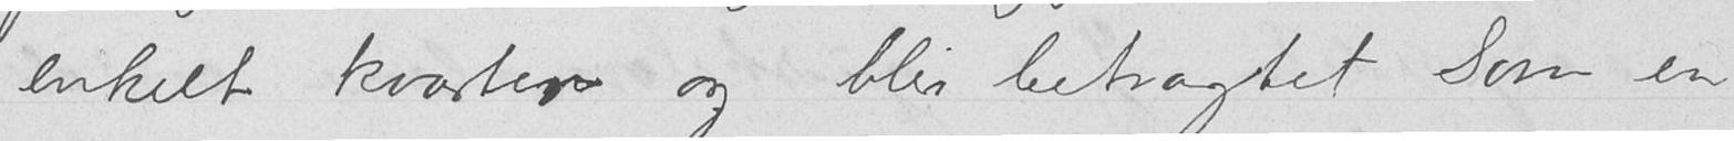enkelt kvarter og blir betragtet som en
